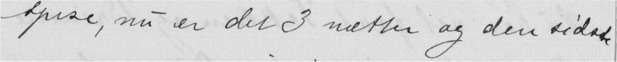spise, nu er det 3 nætter og den sidste
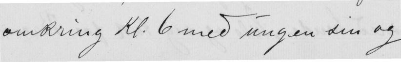omkring Kl. 6 med ungen sin og
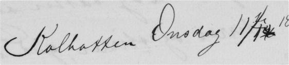Kolhatten Onsdag 11/48
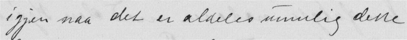igjen naa det er aldeles umulig dette
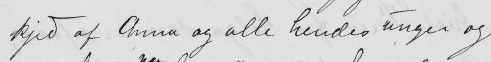kjed af Anna og alle hendes unger og
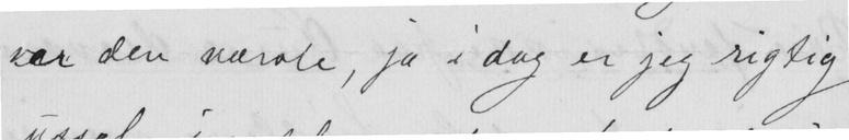var den værste, ja idag er jeg rigtig
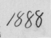1888
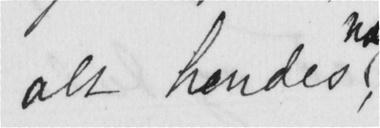alt hendes,
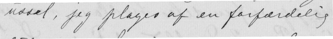ussel, jeg plages af en forfærdelig
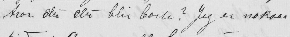tror du du blir borte? Jeg er noksaa
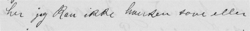her jeg kan ikke hverken sove eller
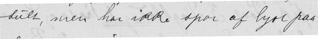sult, men har ikke spor af Lyst paa
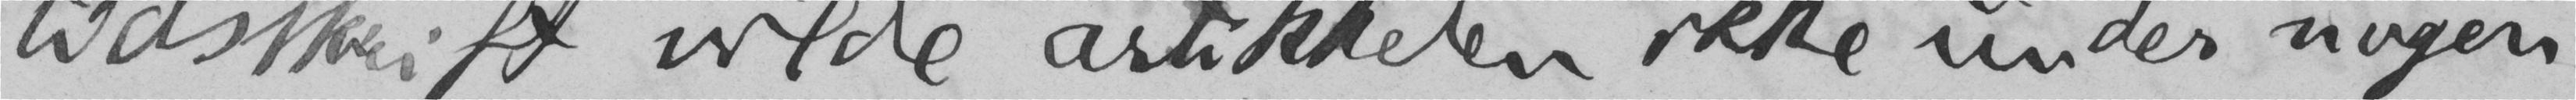tidsskrift vilde artikkelen ikke under nogen
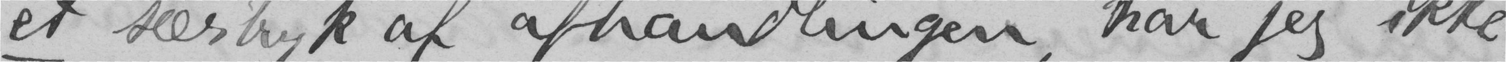et særtryk af afhandlingen, har jeg ikke
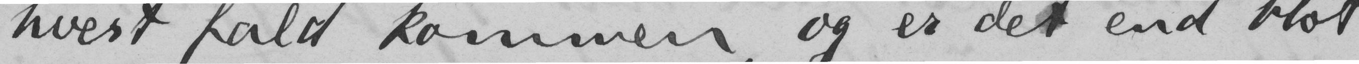hvert fald kommen, og er det end blot
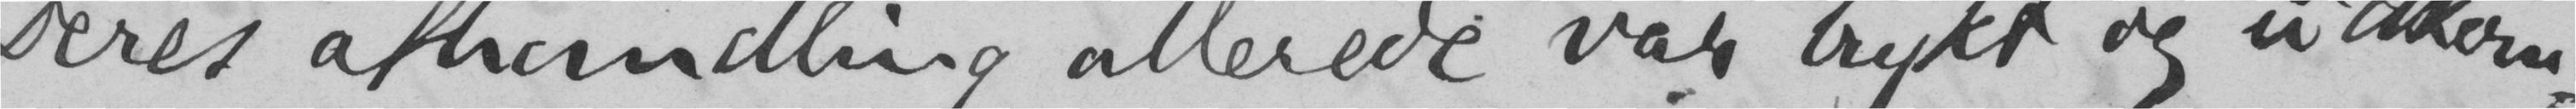Deres afhandling allerede var trykt og udkom-
